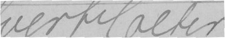Iver Holter
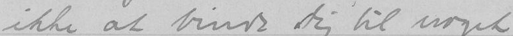ikke at binde dig til noget
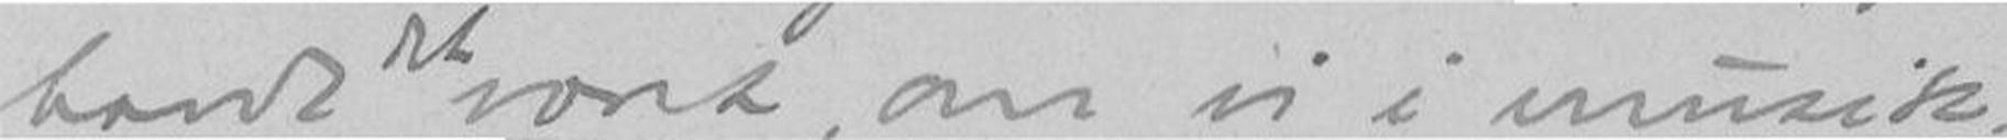havde det været, om vi i musik-
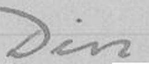Din
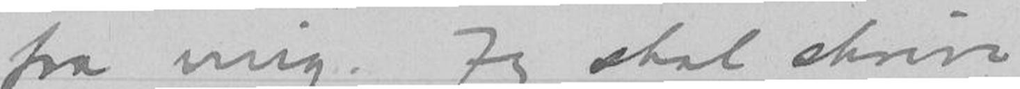fra mig. Jeg skal skrive
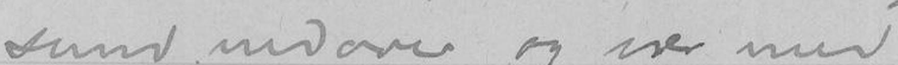sund nedover og vær med
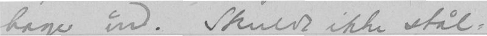bage ind. Skulde ikke stål-
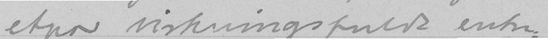etpar virkningsfulde entre-
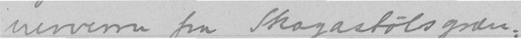nerverne fra Skagastølsgræn-
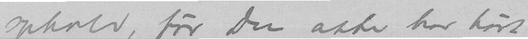ophold, før du atter har hørt
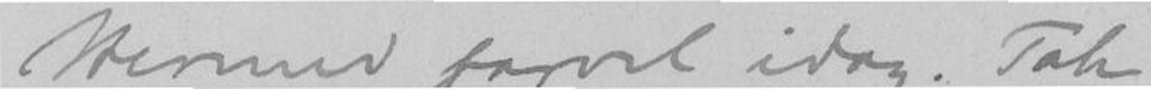Hermed farvel idag. Tak
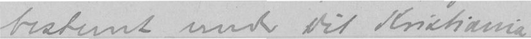bestemt med dit Kristiania
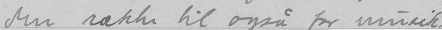den række til også for musik-
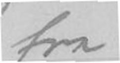fra
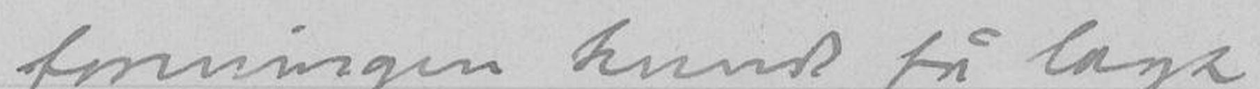foreningen kunde få lagt
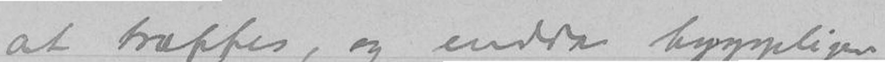at træffes, og endda hyggeligere
